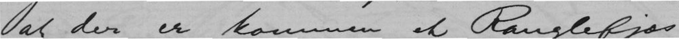at der er kommen et Ranglefjæs
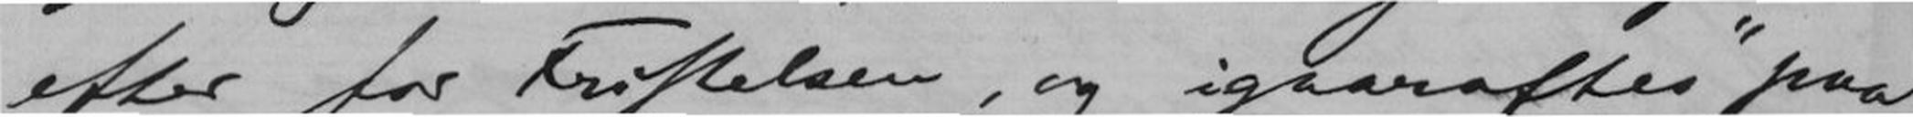efter for Fristelsen, og igaaraftes "paa
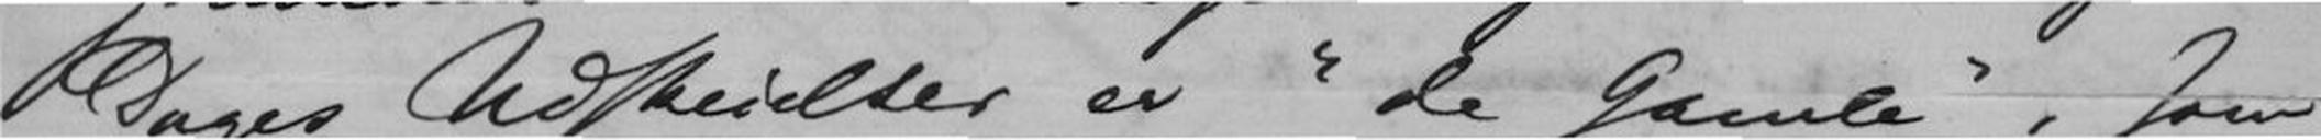Dagers Udskeielser er "de Gamle", som
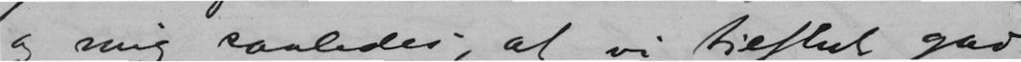og mig saaledes, at vi tilslut gav
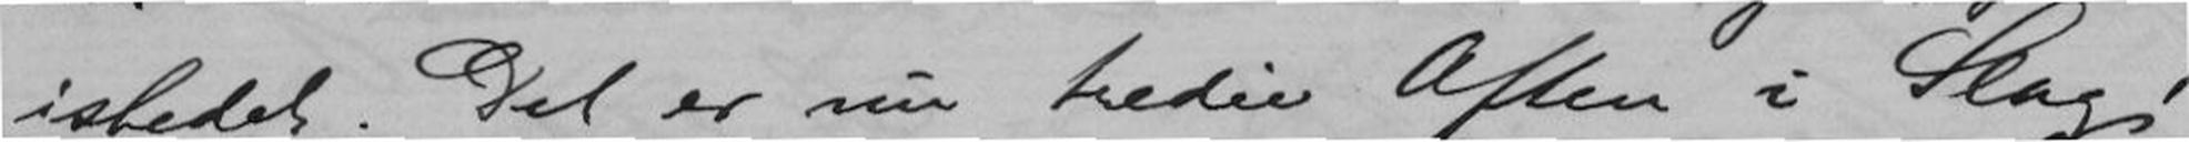istedet. Det er nu tredie Aften i Slags
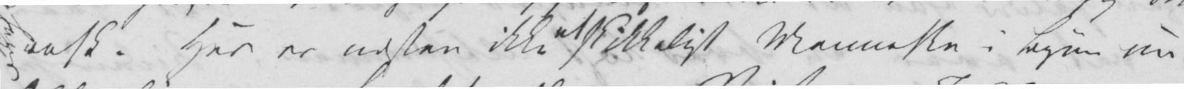rask. Her er næsten ikke et skikkelig Menneske i Byen nu
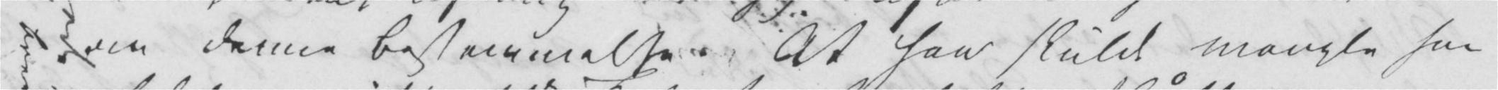om denne Bestemmelse. At han skulde mangle saa
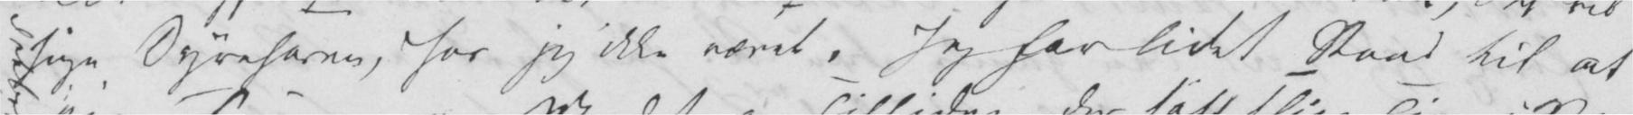sige Dyrehaven, har jeg ikke været. Jeg har lidet Raad til at
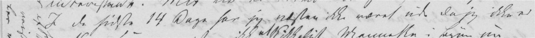I de sidste 14 Dage har jeg næsten ikke været ude da jeg ikke er
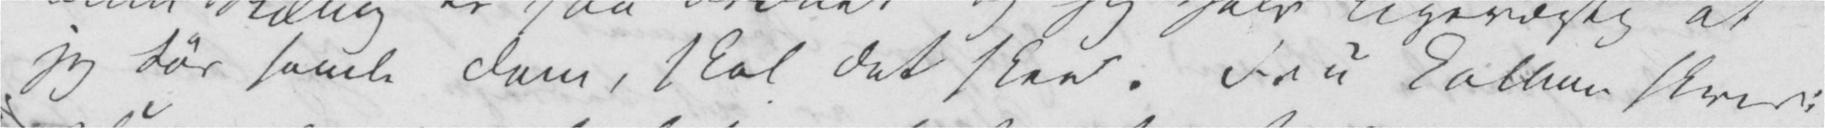jeg tør samle dem, skal det skee. Fru Colban skrev:
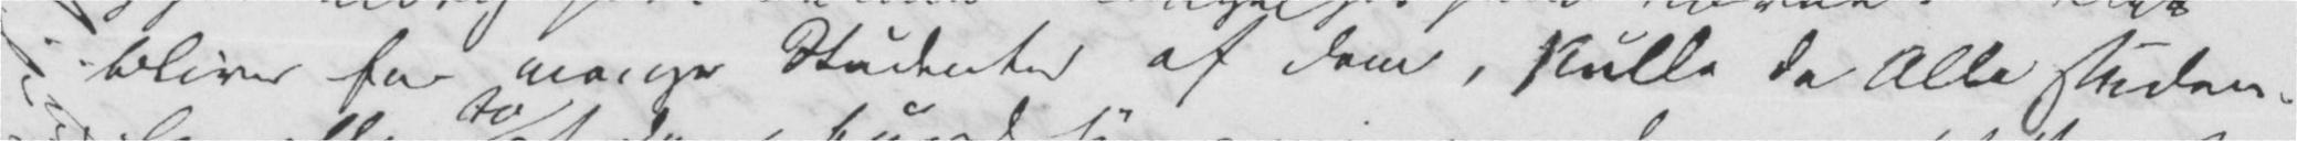bliver for mange Studenter af dem, skulle de Alle studere.
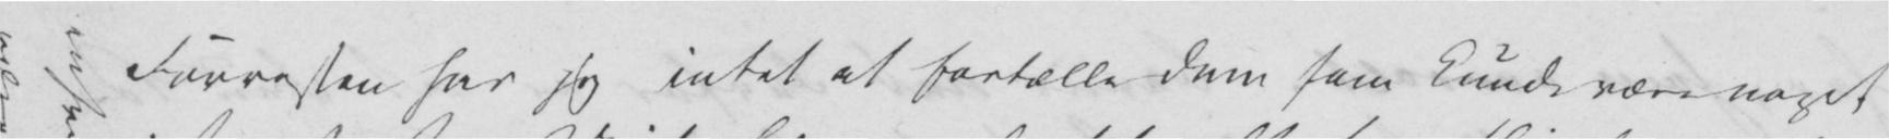Forresten har jeg intet at fortælle Dem som kunde være noget
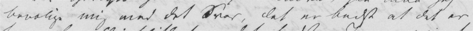berolige mig med det Svar, det er bedst at det er
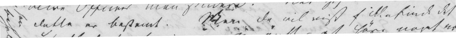dette er bestemt. Men De vil vist f ikke finde det
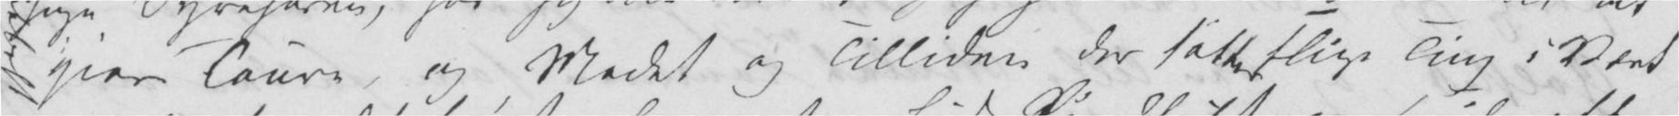gjøre Toure, og Modet og Tilliden der satter slige Ting i Værk
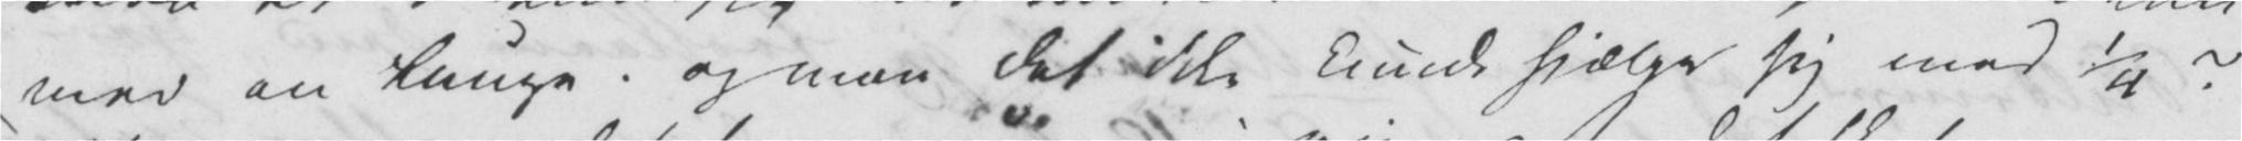med en Lunge. og mon det ikke kunde hjælpe sig med ¼?
